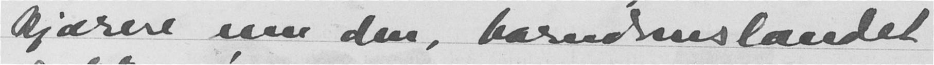kjærere enn den, barndomslandet
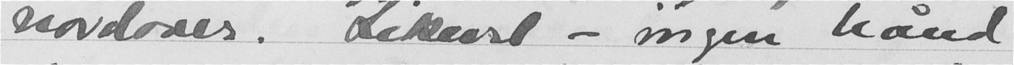nordover. Likevel - ingen hånd,
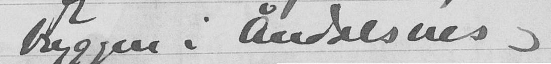bryggen i Åndalsnes og
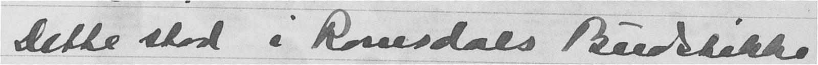Dette stod i Romsdals Budstikke
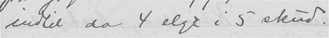indtil da 4 elge i 5 skud.
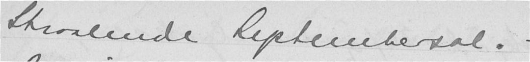Straalende Septembersol.
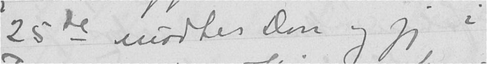25de mødtes Don og jeg i
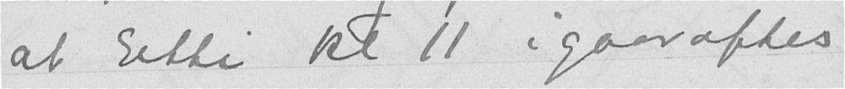at Etti kl 11 i gaar aftes
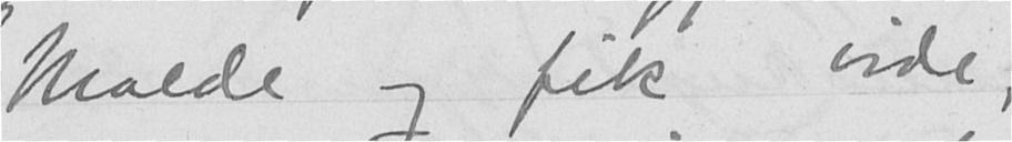Molde og fik vide,
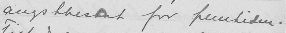angstbesat for fremtiden.
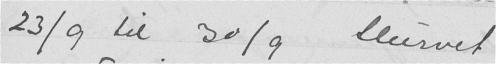23/9 til 30/9 Slurvet
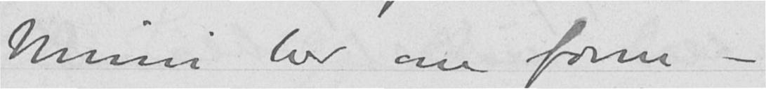Minni her om form -
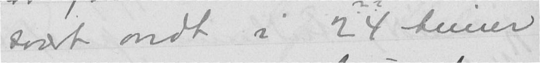svært ondt i 24 timer
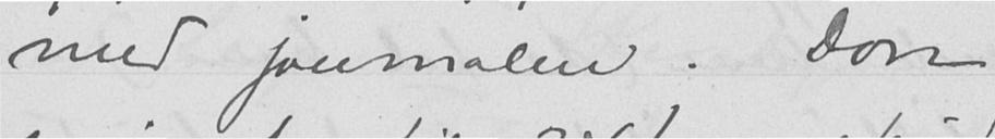med journalen. Don
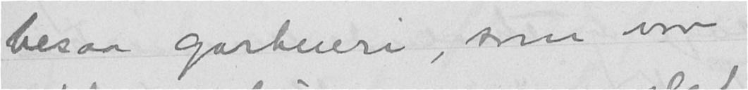besaa gartneri, som var
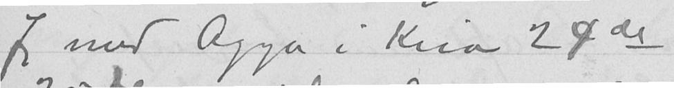Jeg med Agga i Kria 24de
